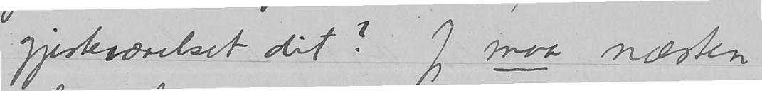gjesteværelset dit? Jeg maa næsten
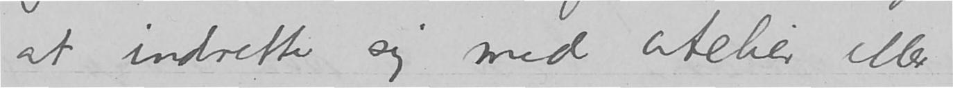at indrette sig med atelier eller
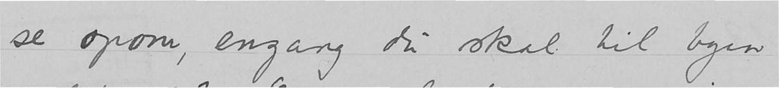se opom, engang du skal til byen
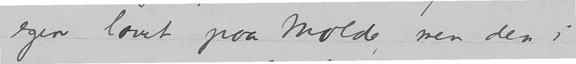egen lavet paa Molde, men det i
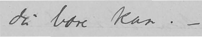du bare kan. -
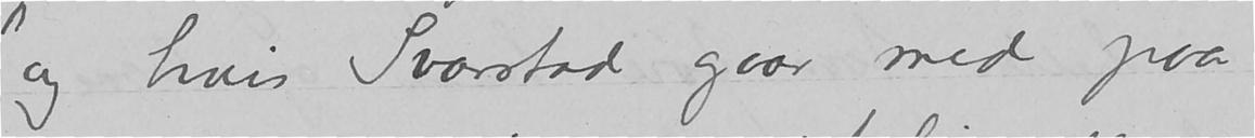og hvis Svarstad gaar med paa
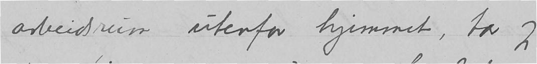arbeidsrum utenfor hjemmet, tar jeg
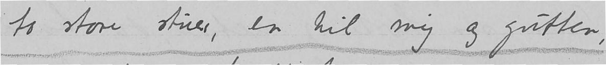to store stuer, en til mig og gutten;
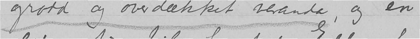grodd og overdækket veranda, og en
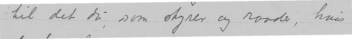til det du som styrer og raader, hvis
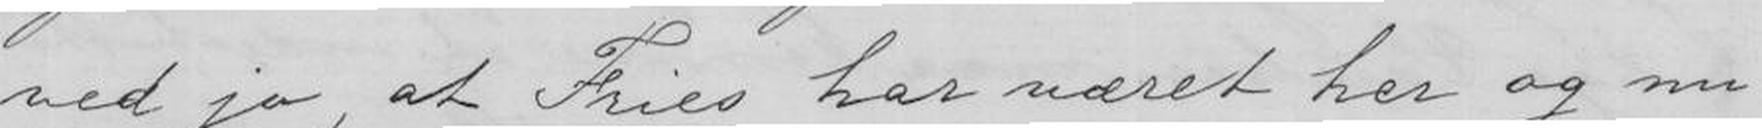ved jo, at Fries har været her og nu
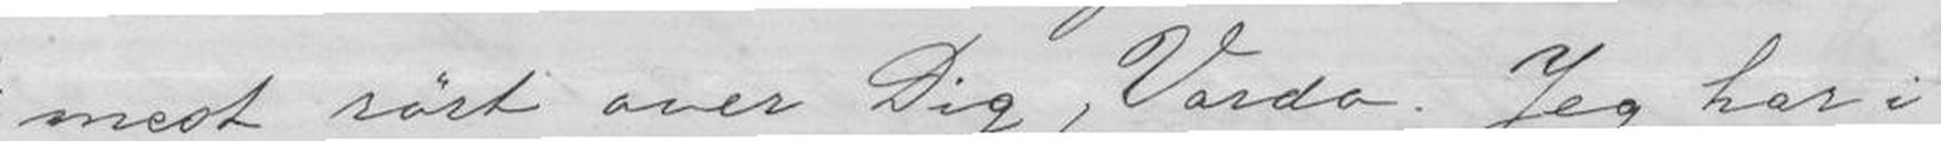mest rørt over Dig, Vardo. Jeg har i
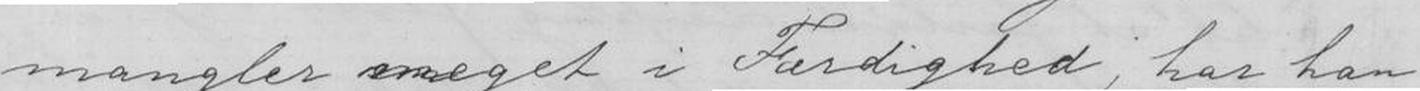mangler meget i Færdighed; har han
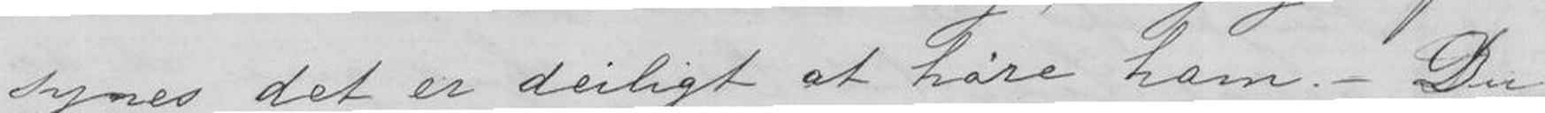synes det er deiligt at høre ham. - Du
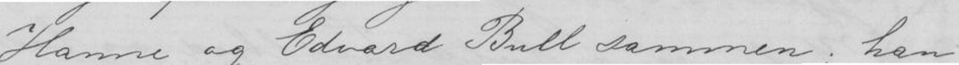Hanne og Edvard Bull sammen; han
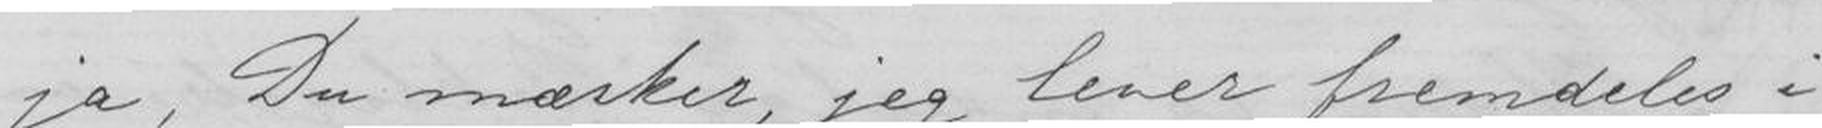- ja, Du mærker, jeg lever fremdeles i
